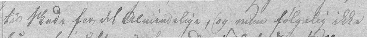til Skade for det Almindelige, og man følgelig ikke
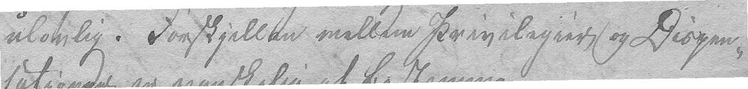ulovlig. Forskjellen mellem Privilegier og Dispen-
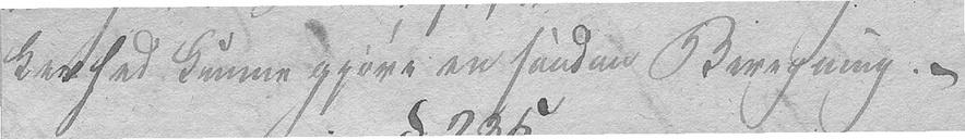kerhed kunne gjøre en saadan Beregning. –
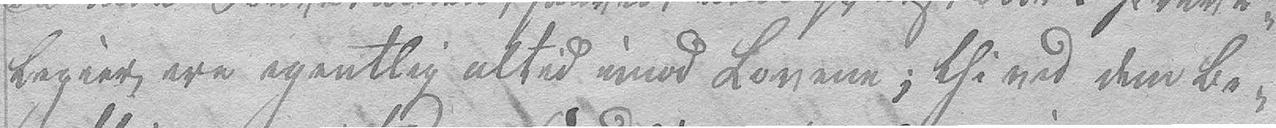legier ere egentlig altid imod Lovene; thi ved dem be-
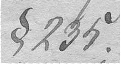§ 235.
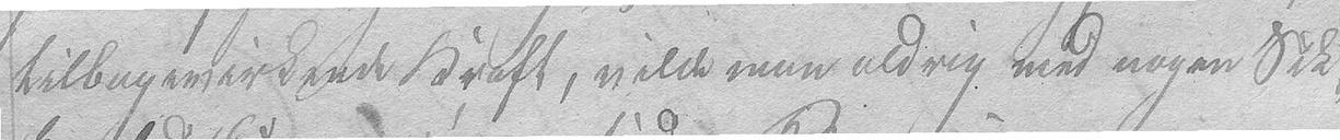tilbagevirkende Kraft, vilde man aldrig med nogen Sik-
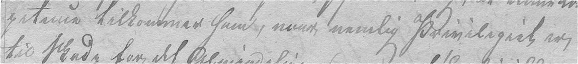petence tilkommer ham, naar nemlig Privilegiet er
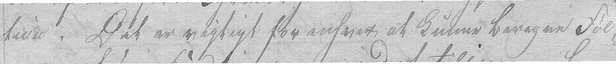tive. Det er vigtigt for enhver at kunne beregne Føl-
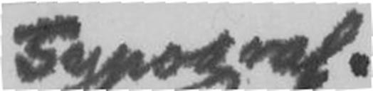Typograf.
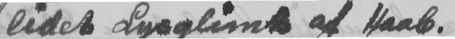lidet Lysglimt af Haab.
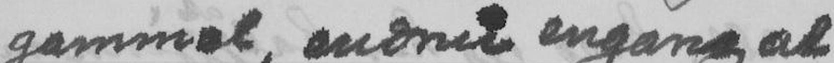gammel, endnu engang at
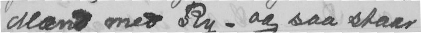Mænd med Ry - og saa staar
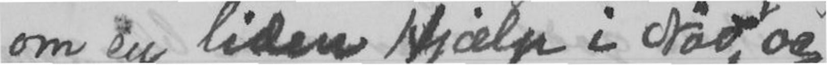om en liden hjælp i Nød, og
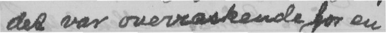det var overraskende for en
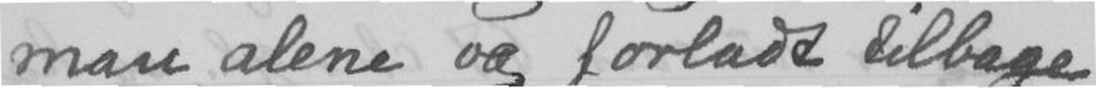man alene og forladt tilbage
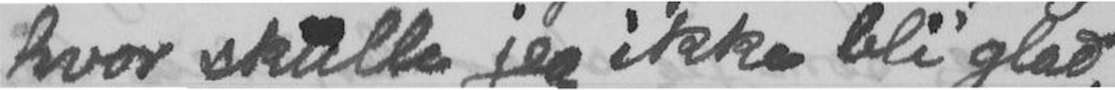hvor skulle jeg ikke bli glad,
